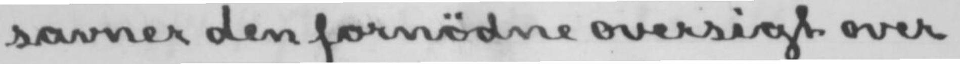savner den fornødne oversigt over
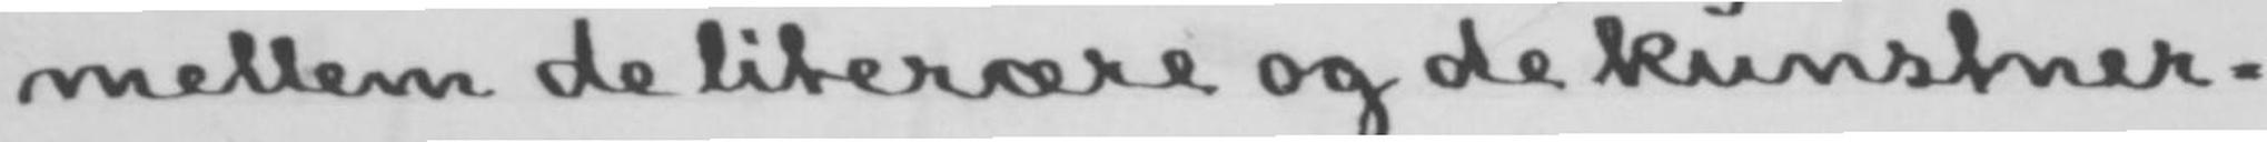mellem de literære og de kunstner-
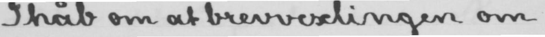I håb om at brevvexlingen om
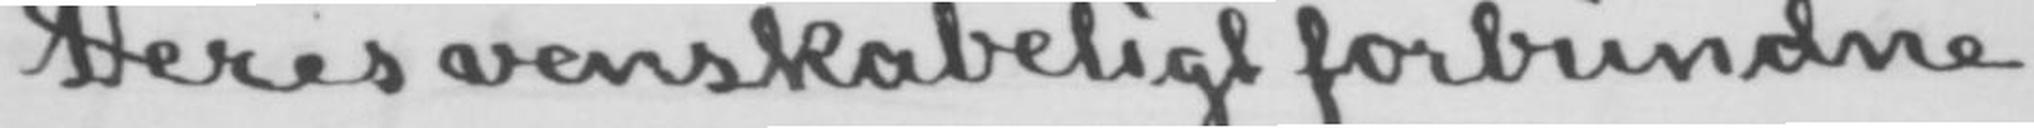Deres venskabeligt forbundne
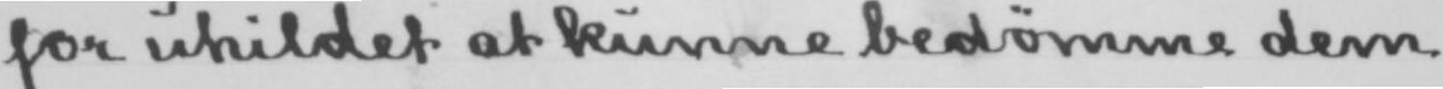for uhildet at kunne bedømme dem
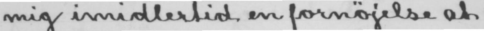mig imidlertid en fornøjelse at
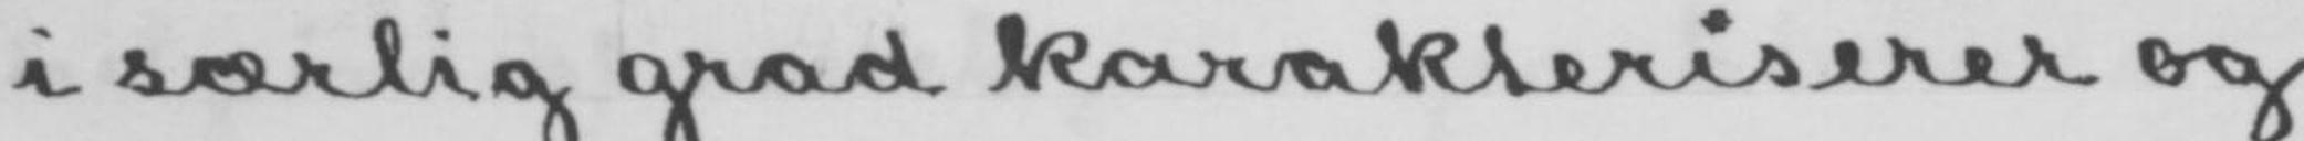i særlig grad karakteriserer og
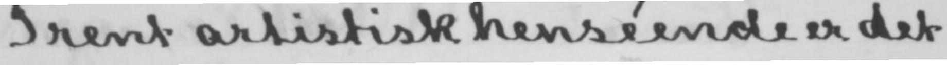I rent artistisk henséende er det
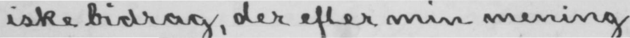iske bidrag, der efter min mening
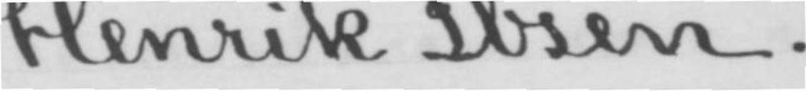Henrik Ibsen.
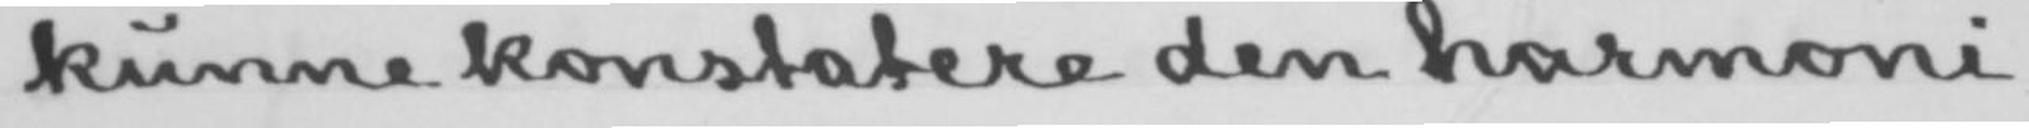kunne konstatere den harmoni
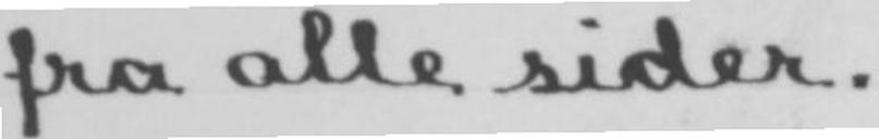fra alle sider.
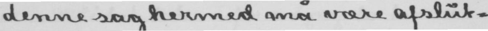denne sag hermed må være afslut-
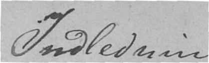Indledning
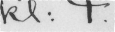kl: 4.
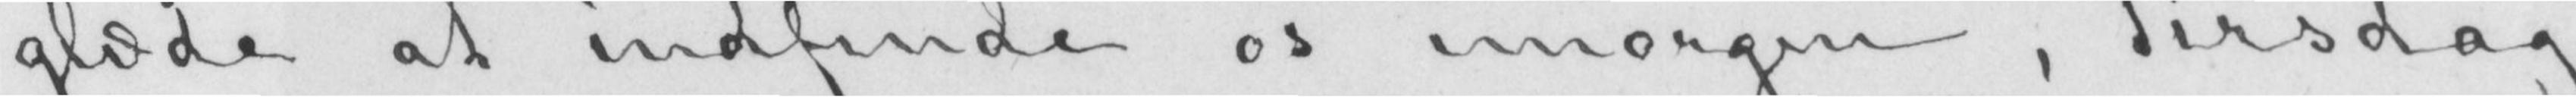glæde at indfinde os imorgen, Tirsdag,
